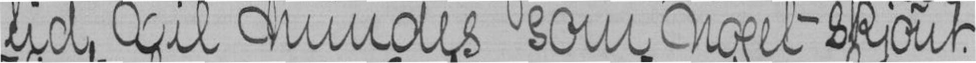tid vil mindes som noget skjønt.
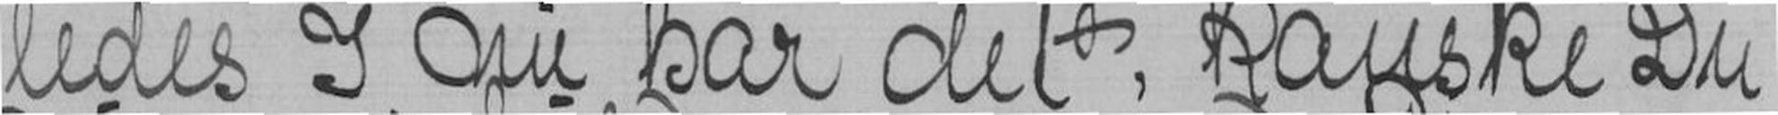ledes I nu har det. Kanske Du
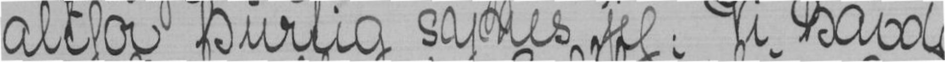altfor hurtig synes jeg. Vi havde
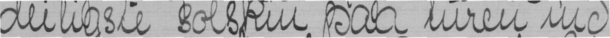deiligste solskin paa turen ind
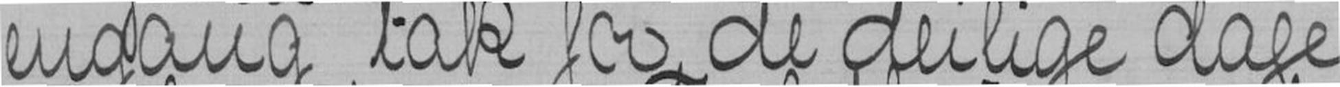engang tak for de deilige dage
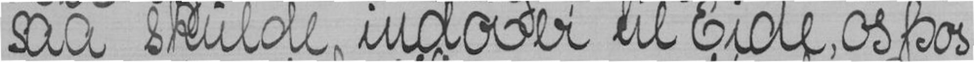saa skulde indover til Eide, øspøs
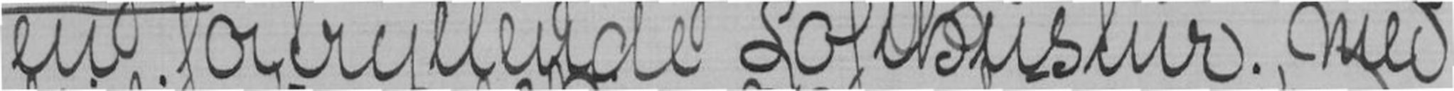en fortryllende Lofthustur. Med
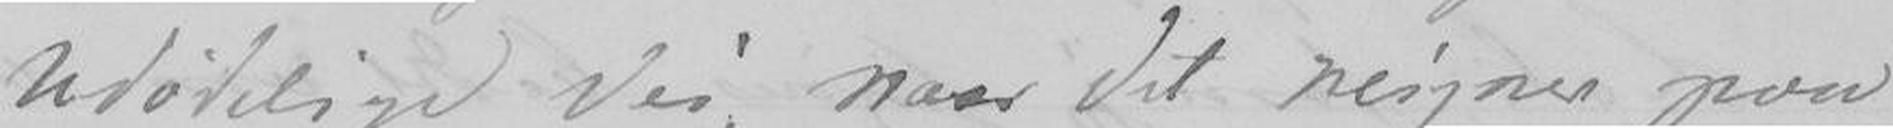Udødelige Vei, naar dit reigner paa
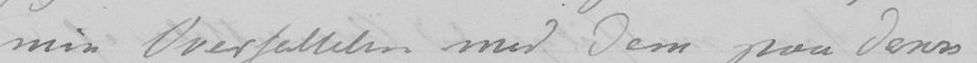min Oversættelse med Dem paa Deres
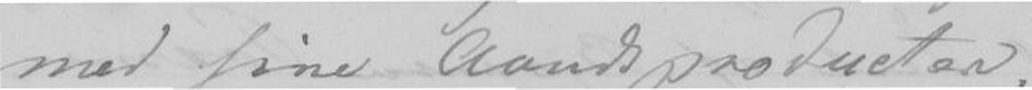med sine Aandssproducter.
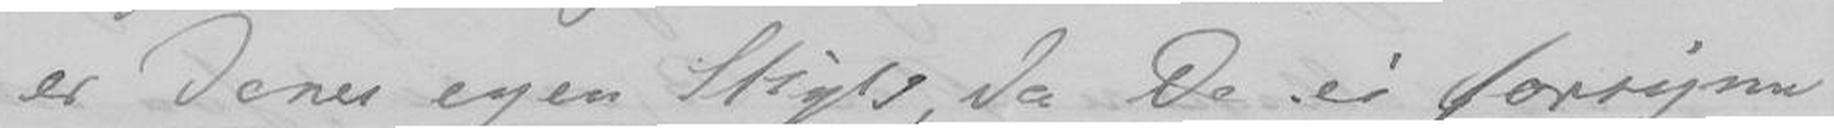er Dere egen skyld, da De ei forrigner
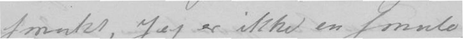smukt, Jeg er ikke en smule
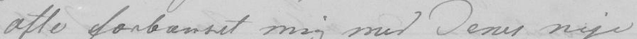ofte forbauset mig med Deres nye
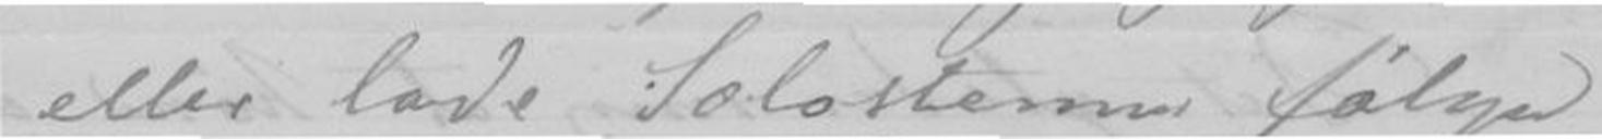eller lade Solostemme følge
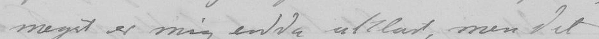meget er mig enda uklart, men det
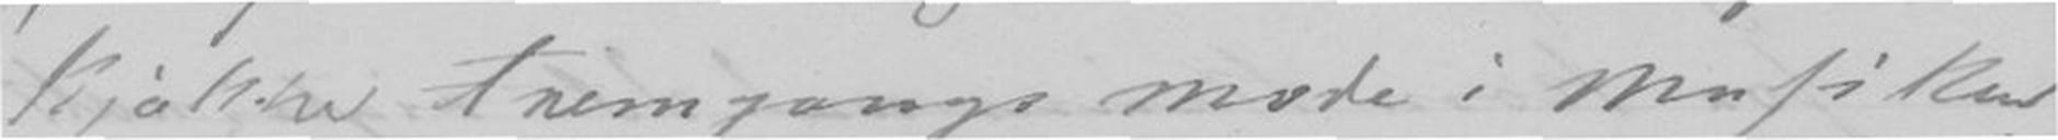kjække fremgange mode o musiken
